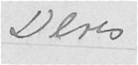Deres
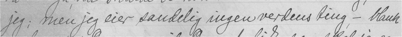jeg; men jeg eier sandelig ingen verdens ting, - blank
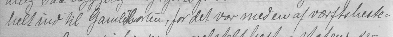helt ind til Gamlehorten, for det var med en af værftsheste-
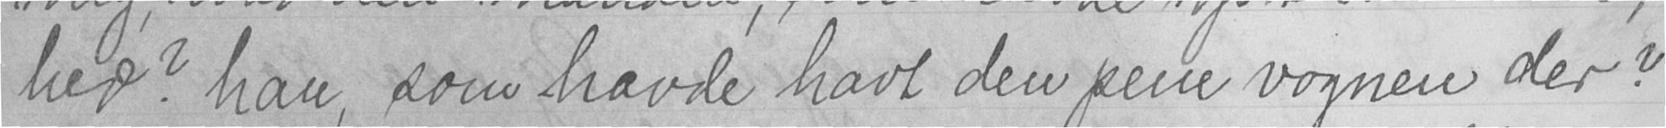hed? han, som havde havt den pene vognen der?
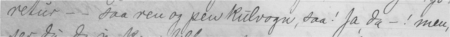retur - - saa ren og pen kulvogn, saa! Ja, da - ! men,
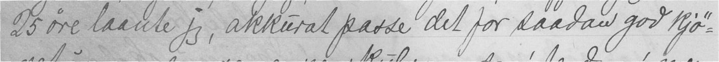25 øre laante jeg, akkurat passe det for saadan god kjø-
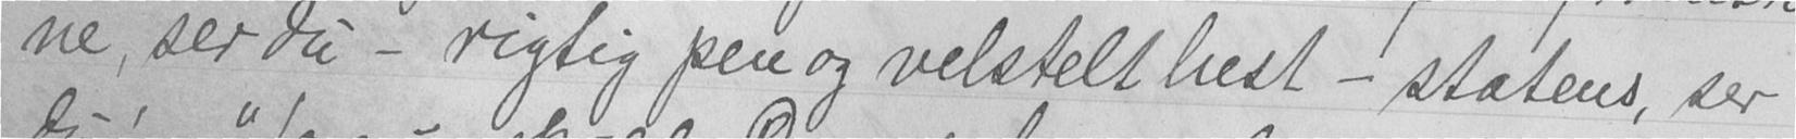ne, ser du - rigtig pen og velstelt hest - statens, ser
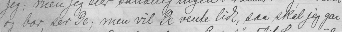og bar, ser De; men vil De vente lidt, saa skal jeg gaa
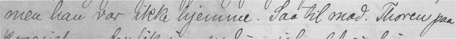men han var ikke hjemme. Saa til mad. Thoren paa
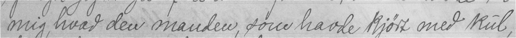mig, hvad den manden, som havde kjørt med kul,
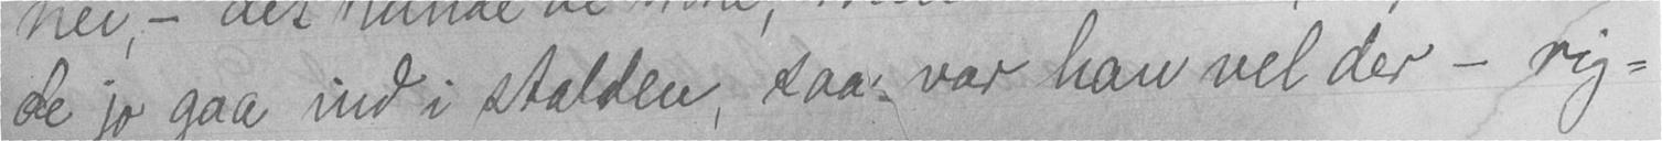de jo gaa ind i stalden, saa var han vel der - rig-
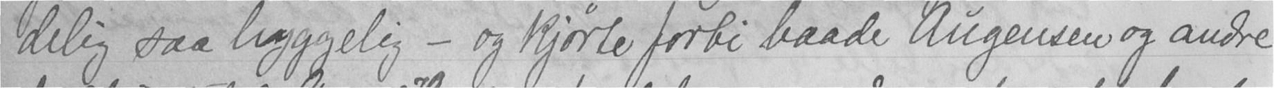deilig saa hyggelig - og kjørte forbi baade Augensen og andre
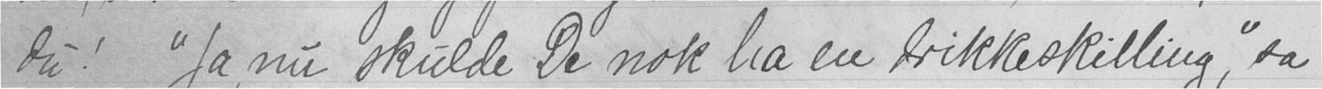du! "Ja, nu skulde De nok ha en drikkeskilling," sa
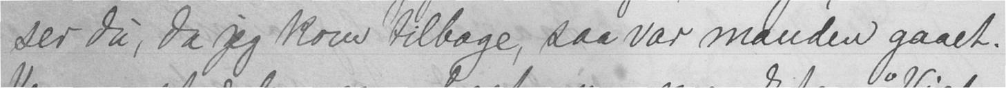ser du, da jeg kom tilbage, saa var manden gaaet.
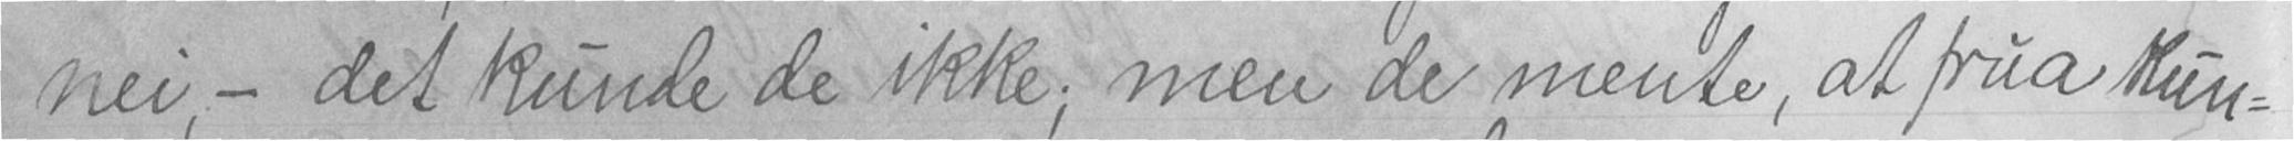nei, - det kunde de ikke; men de mente, at frua kun-
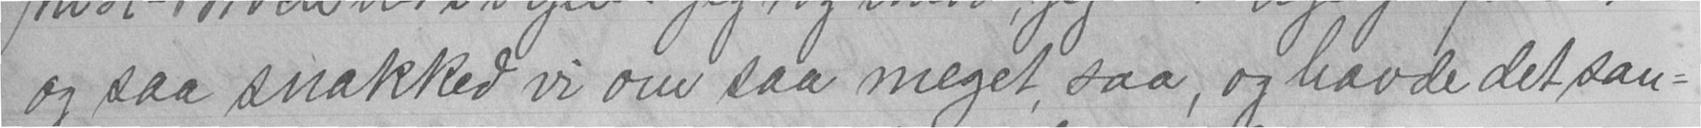og saa snakked vi om saa meget, saa, og havde det san-
